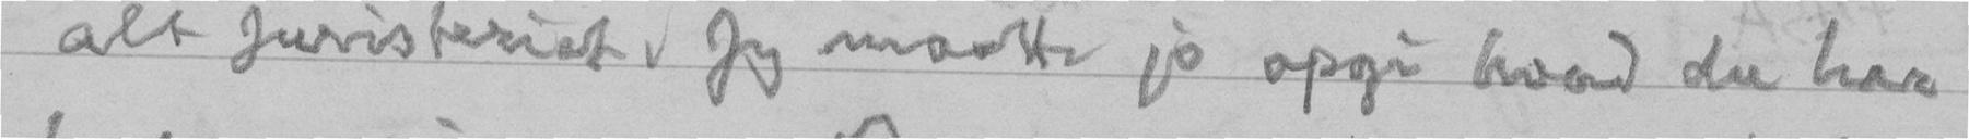alt Juristeriet. Jeg maatte jo opgi hvad du har
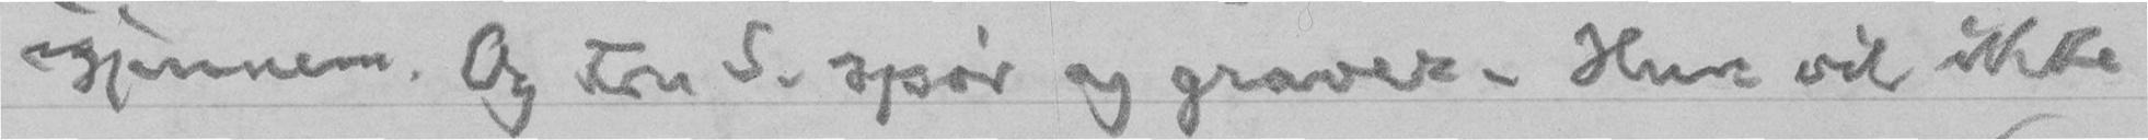igjennem. Og Fru S. spør og graver. Hun vil ikke
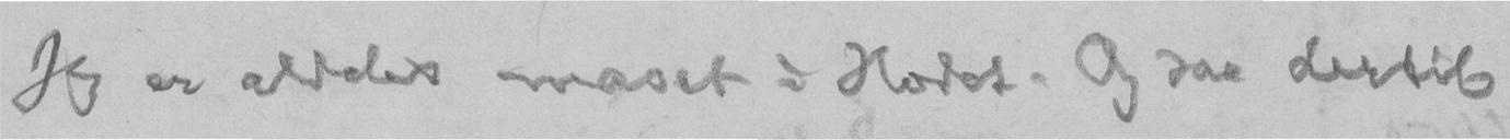Jeg er aldeles maset i Hodet. Og saa dertil
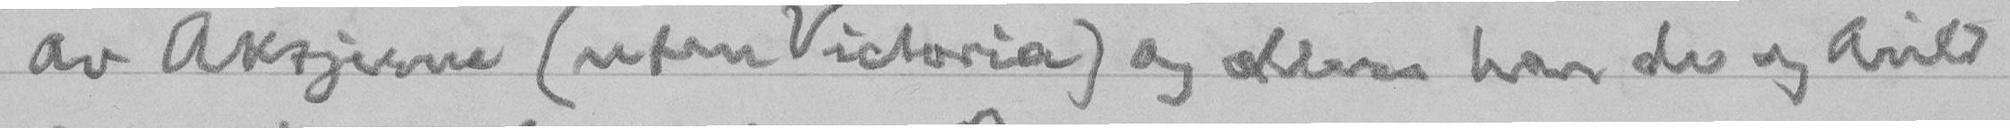av Aksjerne (uten Victoria) og dem har du og Arild
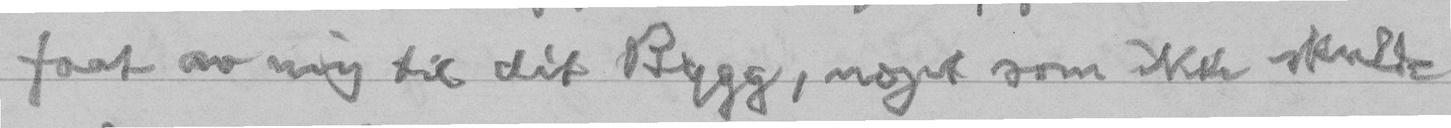faat av mig til dit Bygg, noget som ikke skulde
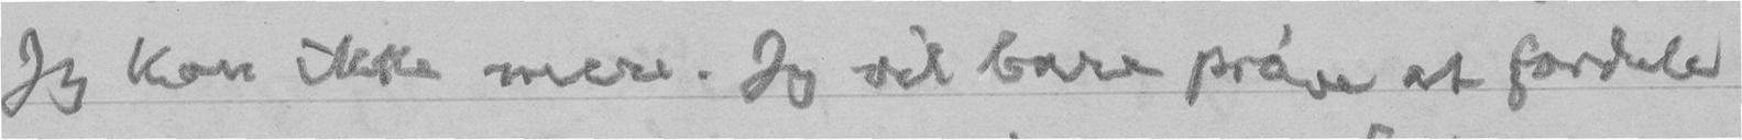Jeg han ikke mere. Jeg vil bare prøve et fordele
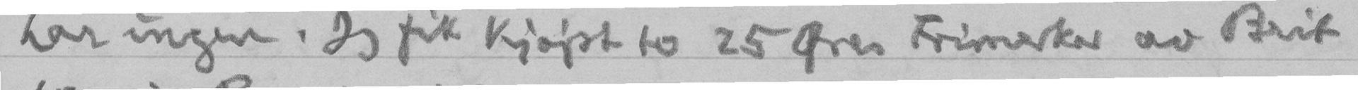har ingen. Jeg fik kjøpt to 25 Øres Frimerker av Brit
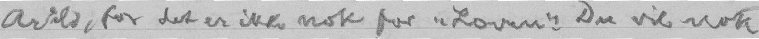Arild, for det er ikke nok for "Loven". Du vil nok
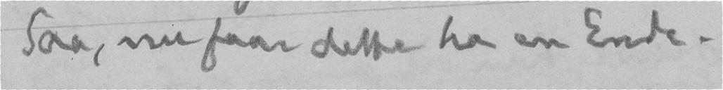Saa, nu faar dette ha en Ende.
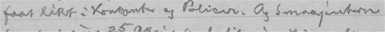faat likt i Kontanter og Policer. Og Smaajenterne
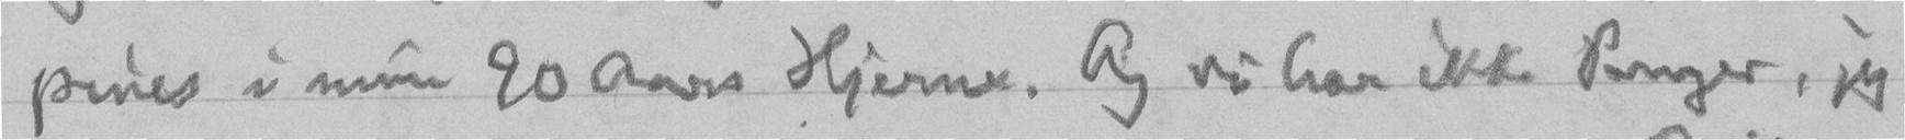pines i min 90 Aars Hjerne. Og vi har ikke Penger, jeg
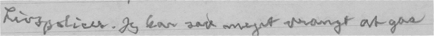Livspolicer. Jeg har saa meget vrangt at gaa
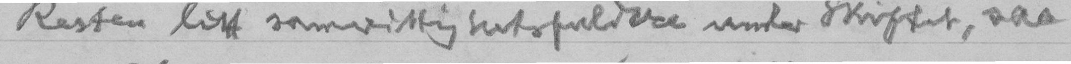Resten litt samvittighetsfuldere under Skiftet, saa
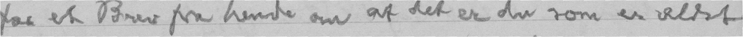faa et Brev fra hende om at det er du som er ældst
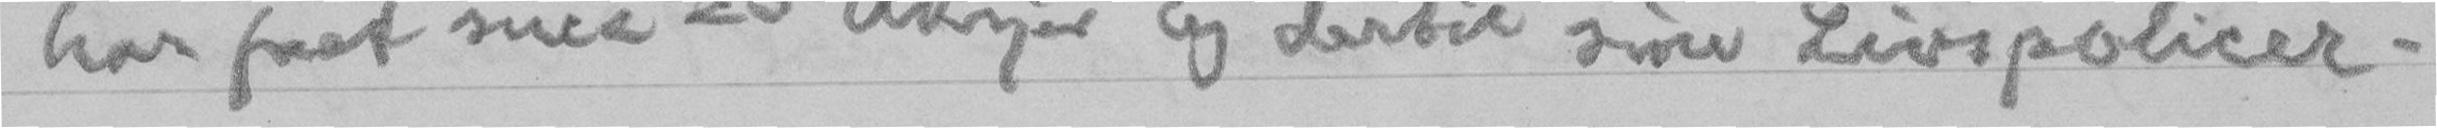har faat sine 25 Aksjer og dertil sine Livspolicer.
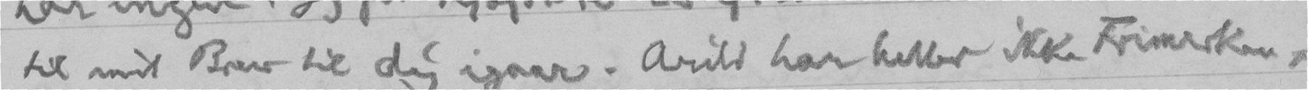til mit Brev til dig igaar. Arild har heller ikke Frimerker,
